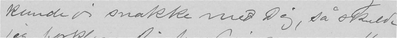kunde vi snakke med Dig, så skulde
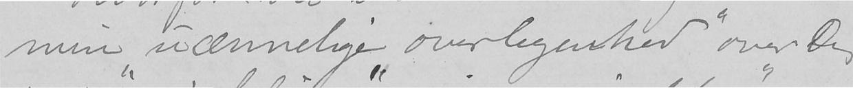min "uænnelige overlegenhed" over Dig
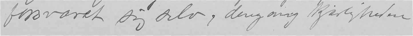forsvaret sig selv; dengang kjærligheden
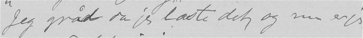Jeg gråd da jeg læste det, og nu er jeg
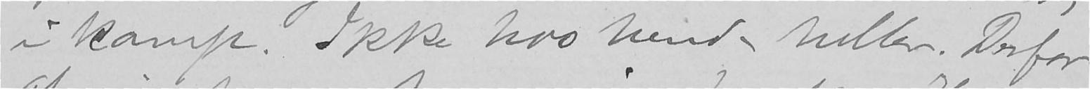i kamp. Ikke hos hende heller. Derfor
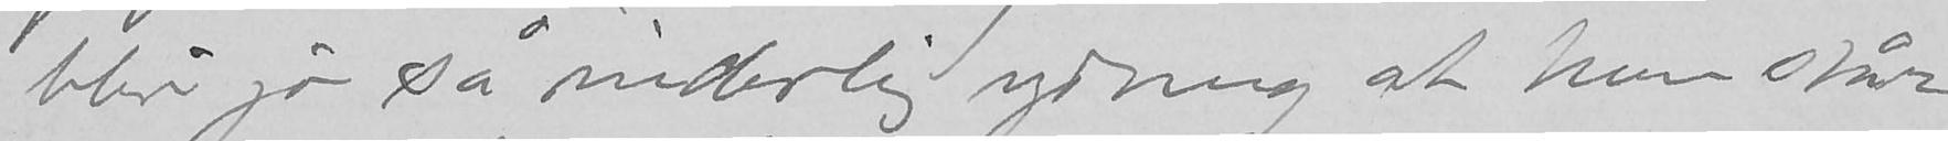blir jo så inderlig ydmyg at hun står
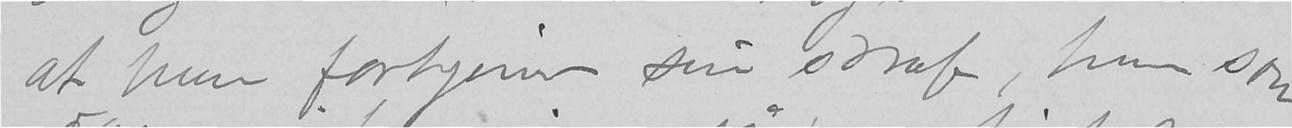at hun fortjener sin straf, hun som
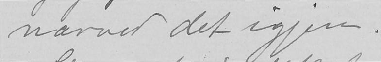nærved det igjen.
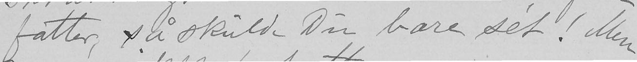fatter, så skulde Du bare sét! Men
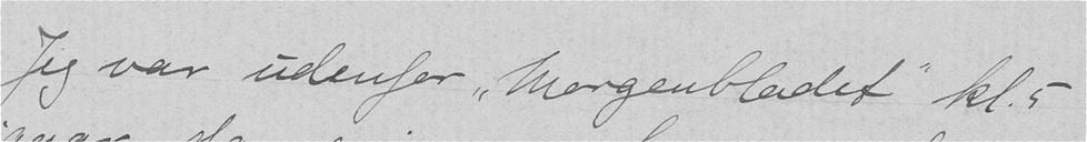Jeg var udenfor "Morgenbladet" kl. 5
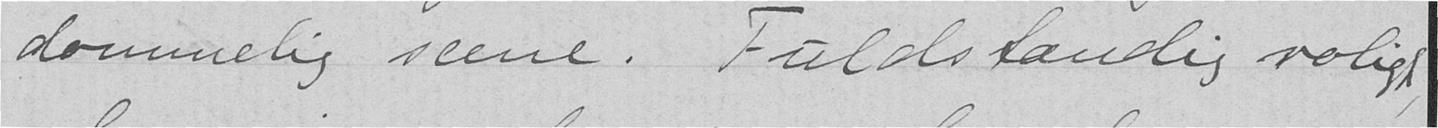dommelig scene. Fuldstændig roligt,
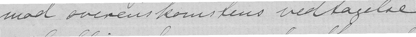mod overenskomstens vedtagelse
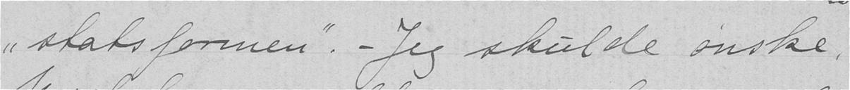"statsformen". - Jeg skulde ønske,
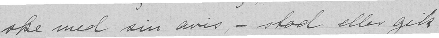ske med sin avis, - stod eller gik
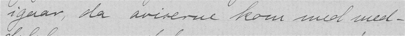igaar, da aviserne kom med med-
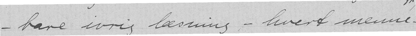- bare ivrig læsning, - hvert menne-
